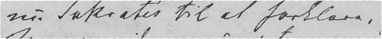nu Sokrates til at forklare,
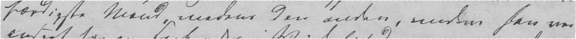færdigste Mand, medens den anden, medens han var
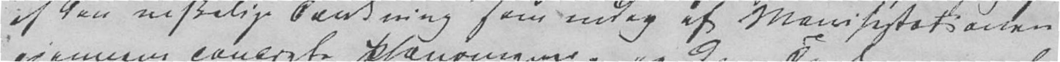af den virkelige Tænkning som endog af Manifestationen
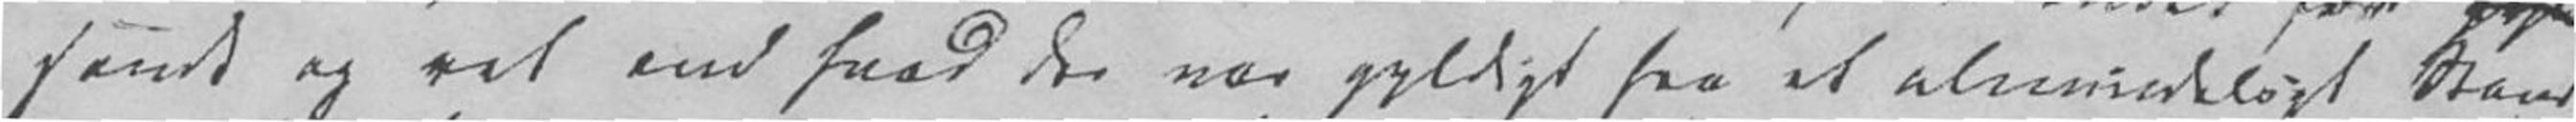sandt og ret end hvad der var gyldigt fra et almindeligt Stand-
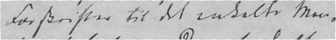Forskrifter til det enkelte Men-
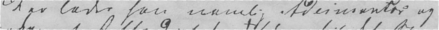Der lader han nemlig Ødeimenter og
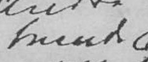X
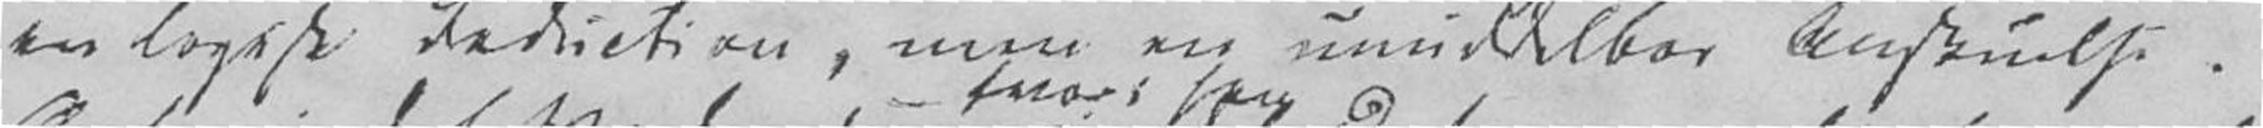en logisk Deduction, men en umiddelbar Anskuelse.
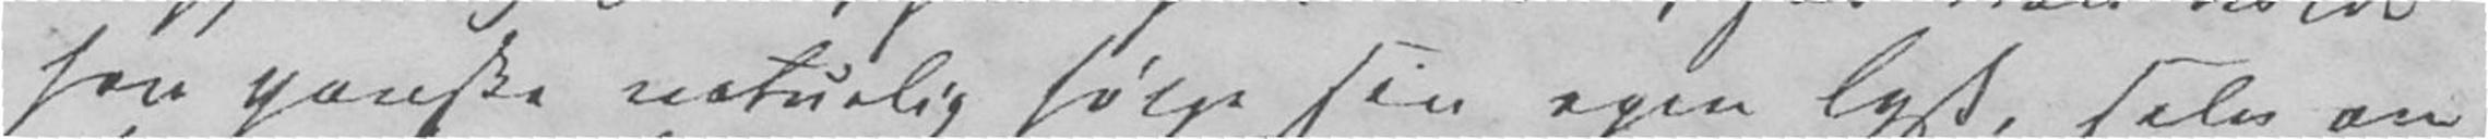han ganske naturlig følge sin egen Lyst, selv om
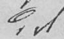det
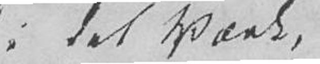i det Værk,
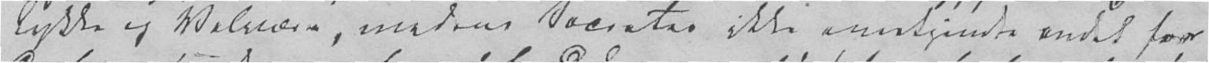Lykke og Velvære, medens Socrates ikke anerkjendte andet for
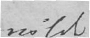vilde
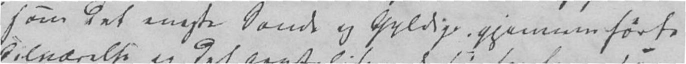som det eneste Sande og Gyldige gjennemførte
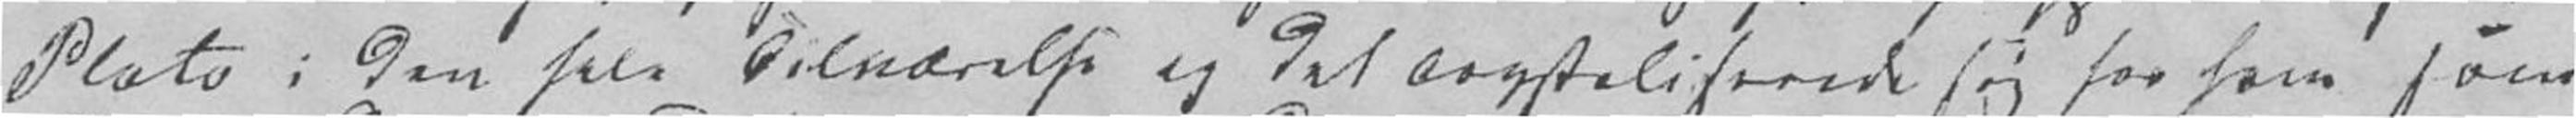Plato i den hele Tilværelse og det lægsteliserede sig for ham som
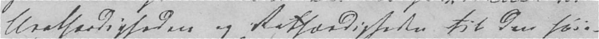Uretfærdigheden og Retfærdigheden til den høie-
